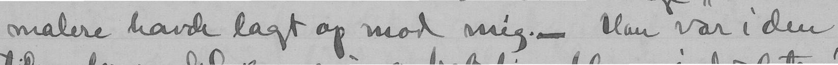malere havde lagt op mod mig. - Han var i den
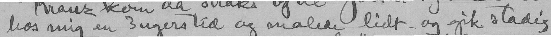hos mig en 3 ugers tid og malede lidt - og gik stadig
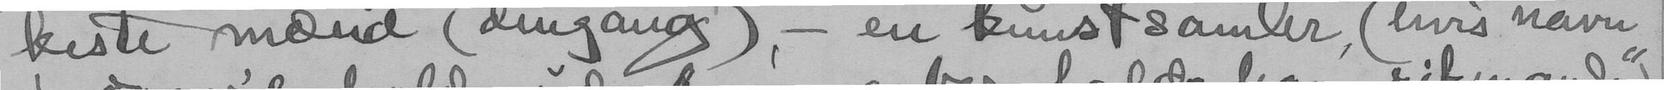kiste mænd (dengang) - en kunstsamler, (hvis navn
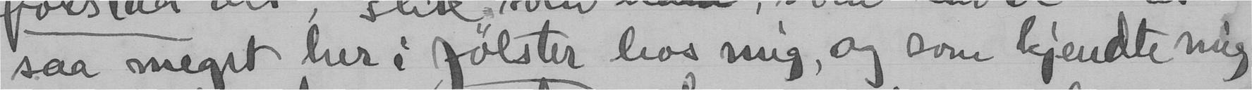saa meget her i Jølster hos mig, og som kjendte mig
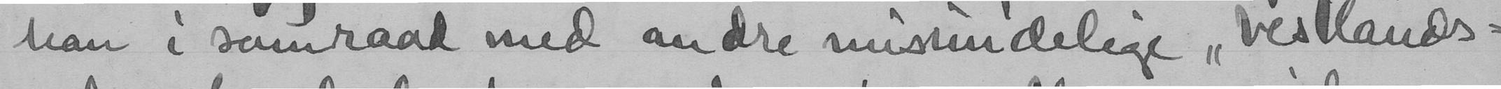han i samraad med andre misundelige "vestlands-
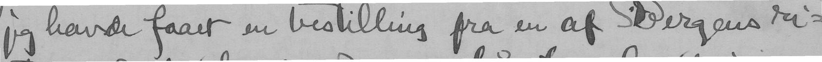jeg havde faaet en bestilling fra en af Bergens ri-
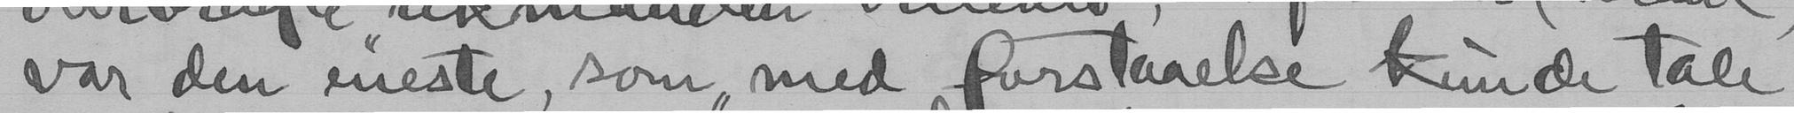var den eneste, som "med forstaaelse kunde tale
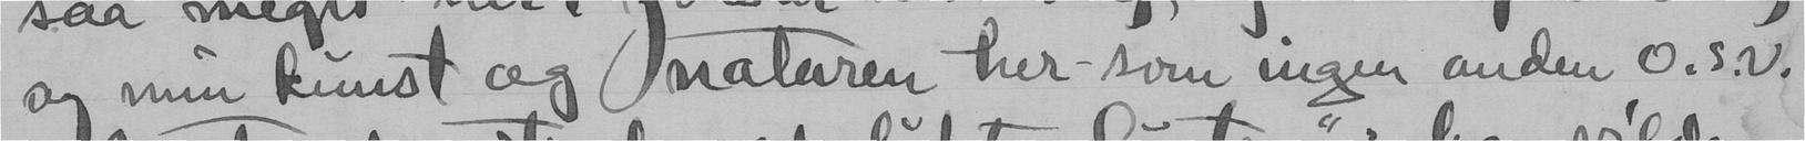og min kunst og naturen her - som ingen anden o.s.v.
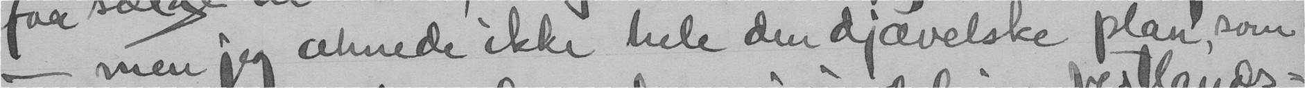- men jeg ahnede ikke hele den djævelske plan, som
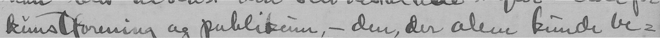kunstforening og publikum, - den, der alene kunde be-
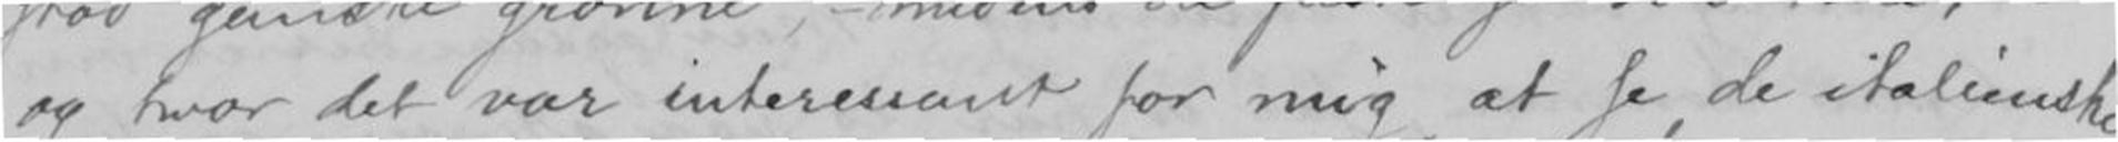og hvor det var interessant for mig at se de italienske
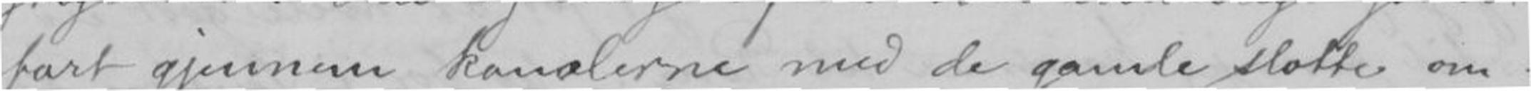fart gjennem kanalerne med de gamle slotte om-
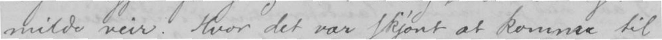milde veir. Hvor det var skjønt at komme til
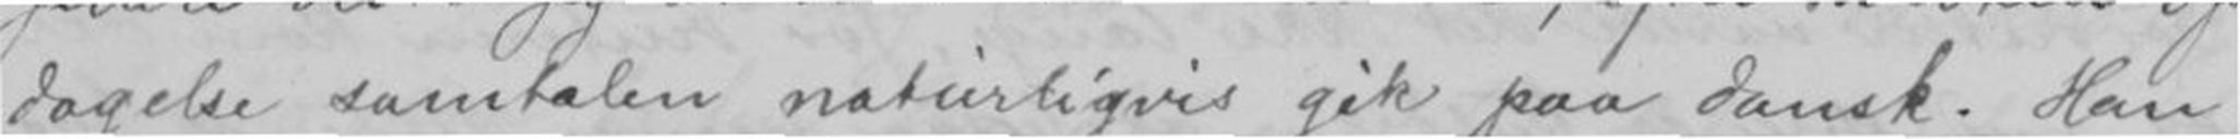dagelse samtalen naturligvis gik paa dansk. Han
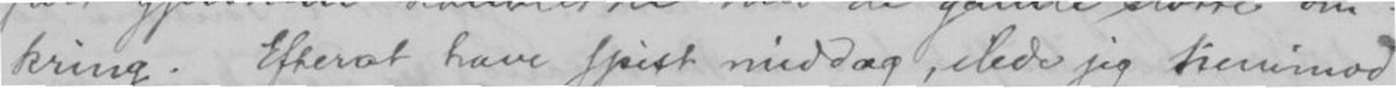kring. Efterat have spist middag, ilede jeg henimod
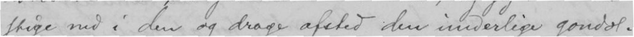stige ned i den og drage afsted den underlige gondol-
